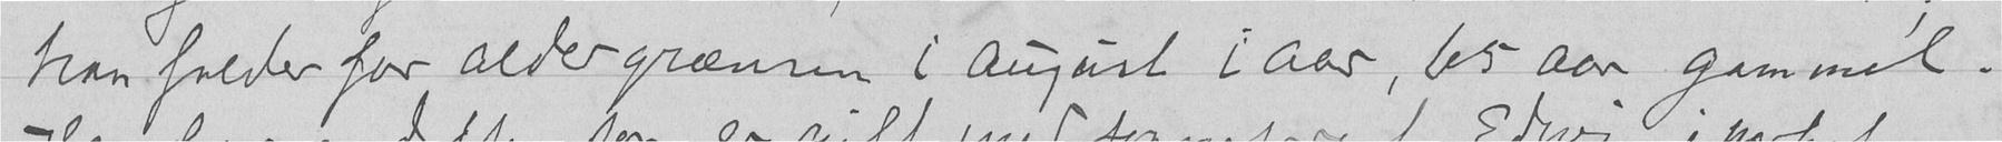han falder for aldergrænsen i august i aar, 65 aar gammel.
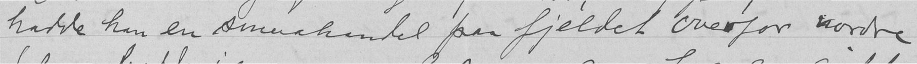hadde han en smaahandel paa fjeldet ovenfor nordre
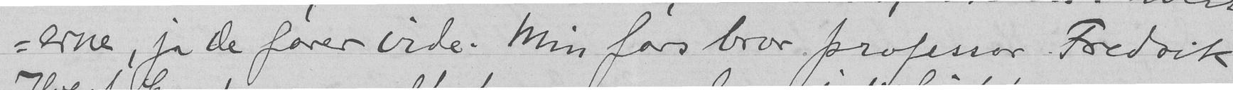erne, ja de farer vide. Min fars bror professor Fredrik
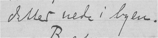datter nede i byen.
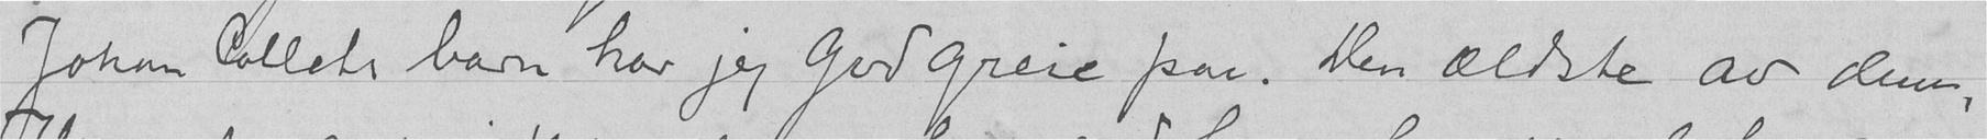Johan Collets barn har jeg god greie paa. Den ældste av dem,
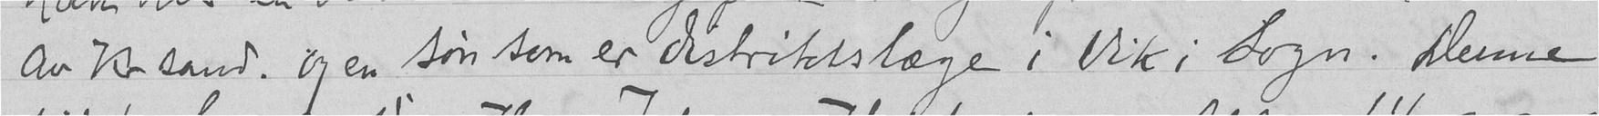av Kr. sand. og en søn som er distriktslæge i Vik i Sogn. Denne
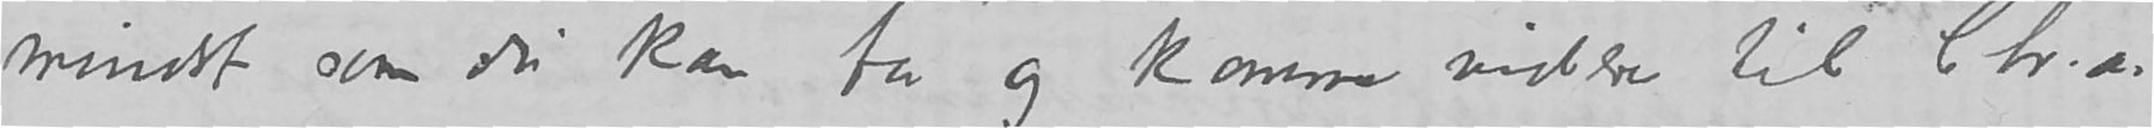mindst som du kan ta og komme videre til Chr.a.
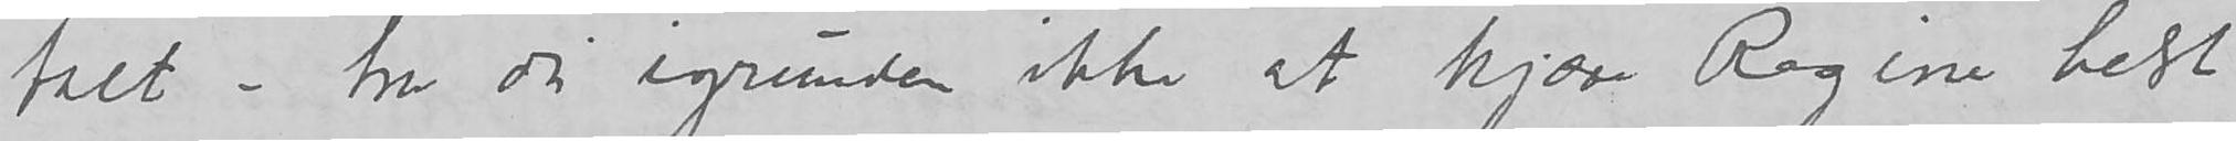talt – tror du igrunden ikke at kjære Regine helst
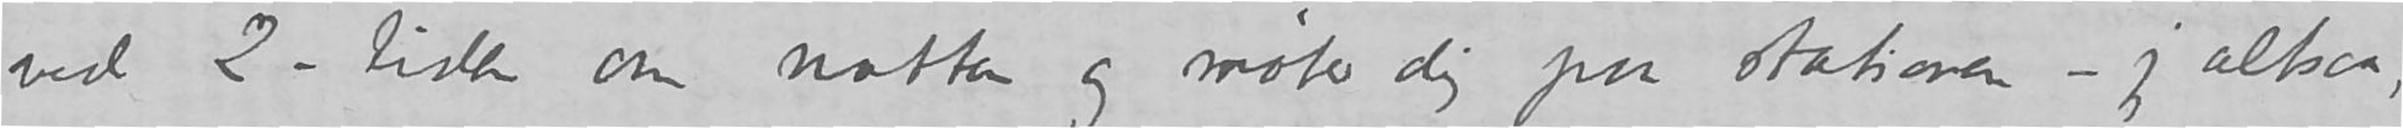ved 2-tiden om natten og møter dig paa stationen – jeg altsaa,
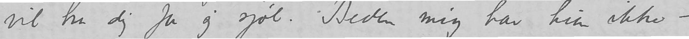vil ha dig for sig sjøl. Beden mig har hun ikke –
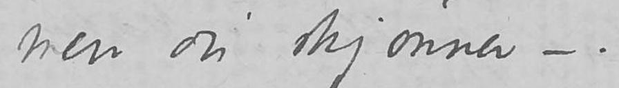men du skjønner –-.
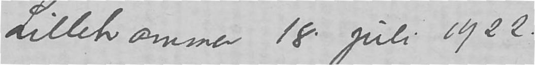Lillehammer 18. juli 1922.
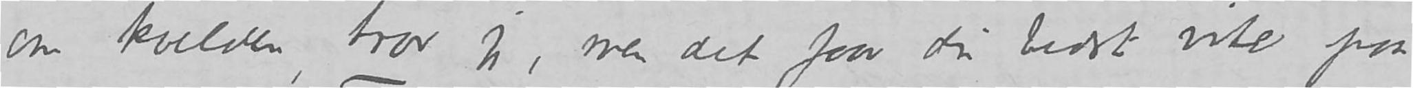om kvelden, tror jeg, men det faar du bedst vite paa
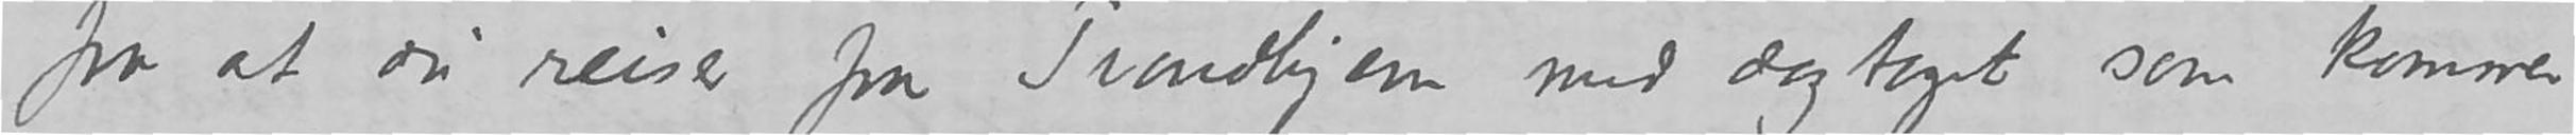at du reiser fra Trondhjem med dagtoget som kommer
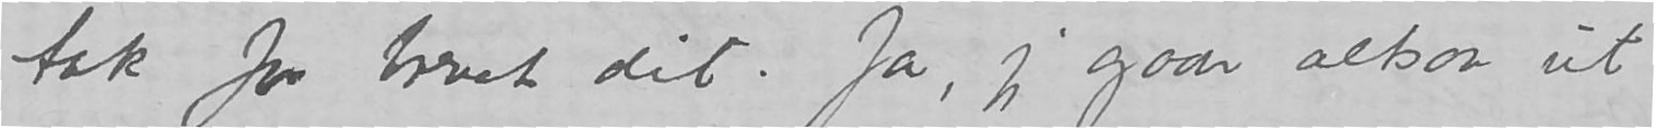tak for brevet dit. Ja, jeg gaar altsaa ut fra
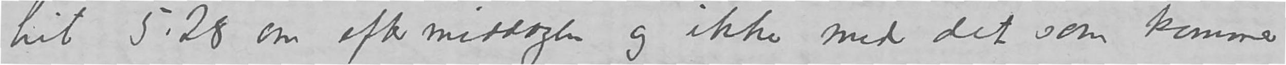hit 5:.28 om eftermiddagen og ikke med det som kommer
